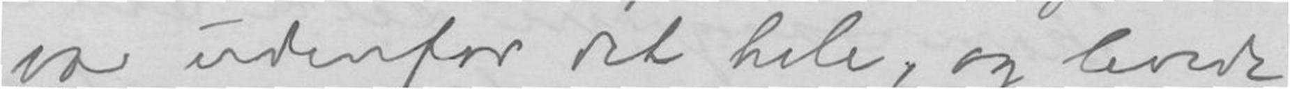var udenfor det hele, og levede
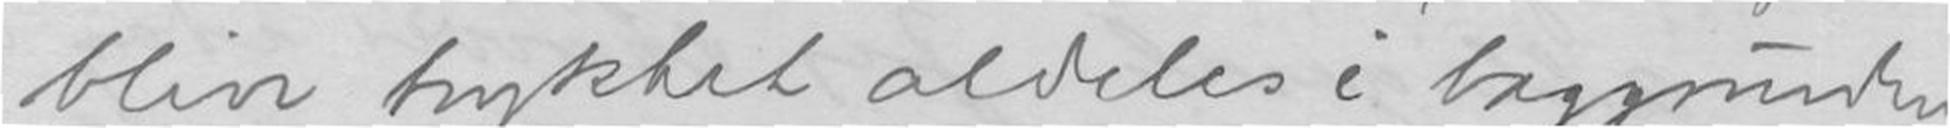blive trykket aldeles i baggrunden
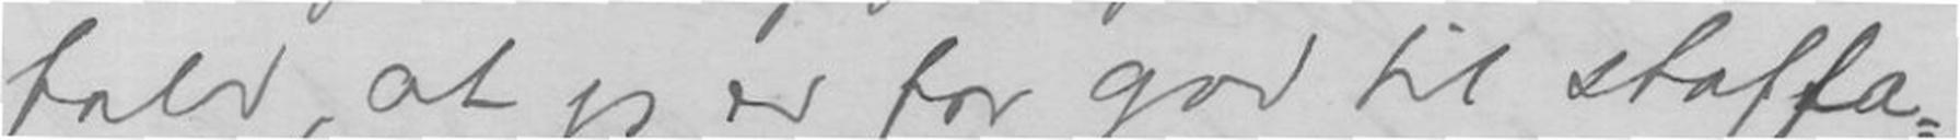fald at jeg er for god til staffa-
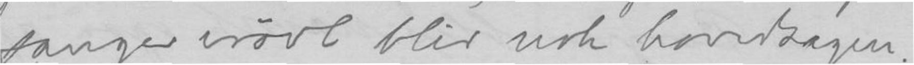sanger vrøvl blir nok hovedsagen.
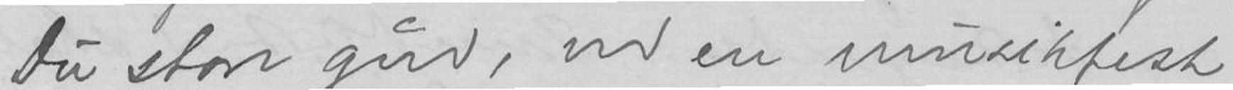Du store gud, med en musikfest
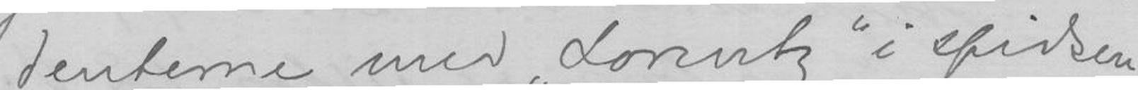denterne med "Lorentz" i spidsen
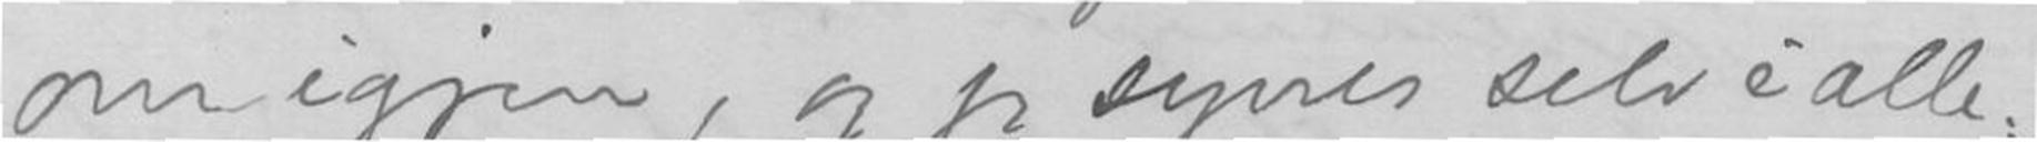om igjen, og jeg synes selv ialle-
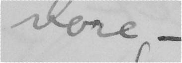vore –
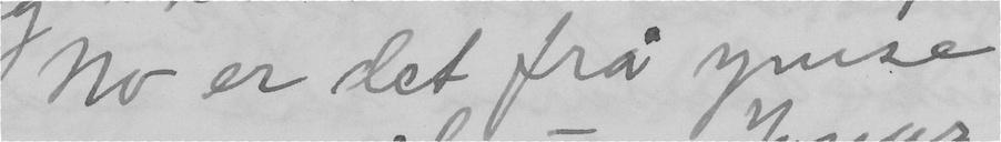No er det frå ymse
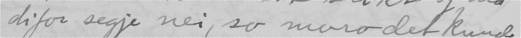difor segje nei, so moro det kunde
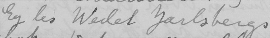Eg les Wedel Jarlsbergs
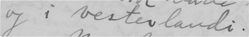og i vesterlandi.
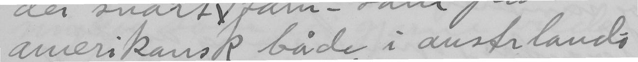amerikansk både i austerlandi
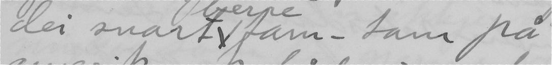dei snart berre tam-tam på
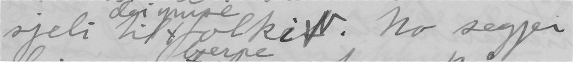sjeli til dei yngre folki. No segjer
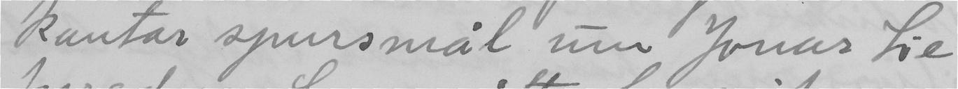kantar spursmål um Jonas Lie
